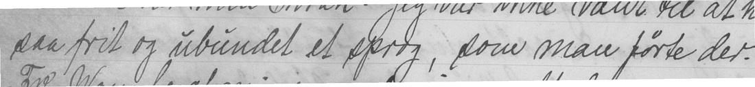saa frit og ubundet et sprog, som man førte der.
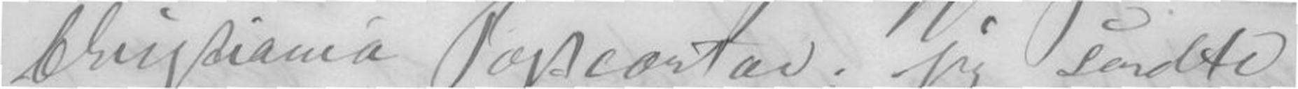Christiania Postcontor; jeg sendte
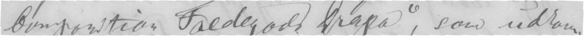Composition Faldegods Drapa, som udkom
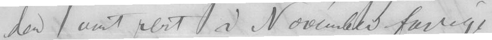den mit post i November forrige
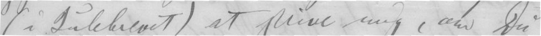(i Julebrevet) at skrive mig, om Du
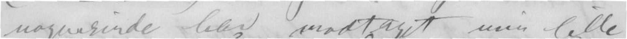nogensinde har modtaget min lille
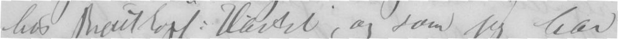hos Breitkopf & Härtel, og som jeg har
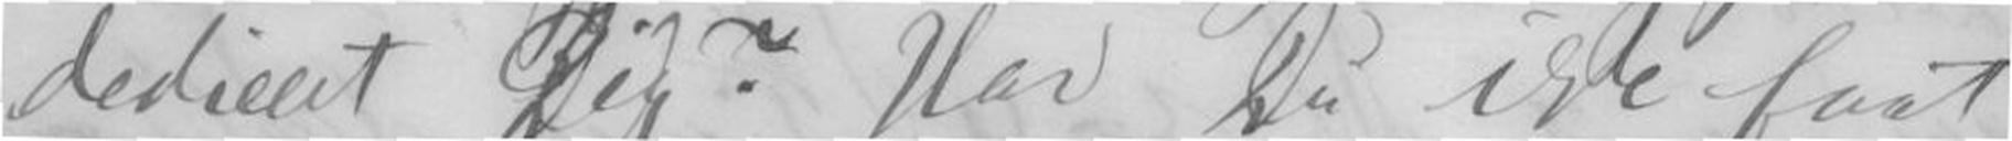dedicert Dig? Har Du ikke faat
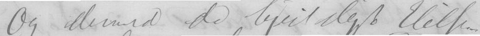Og dermed de hjerteligste Hilse-
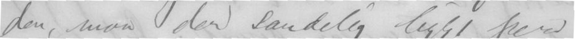den, maa der sandelig ligge paa
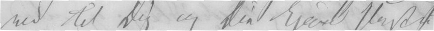ner til Dig og Din kjære Hustru
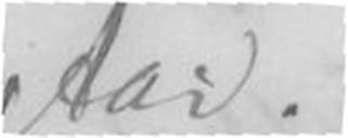Aar.
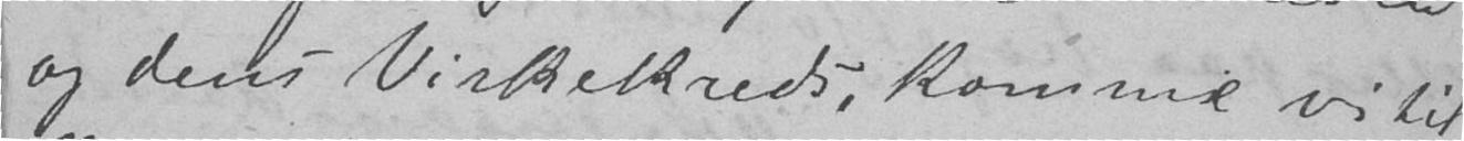og dens Virkekreds, komme vi til
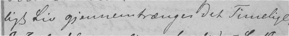ligt Liv gjennemtrænger det Timelige.
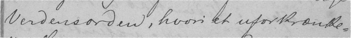Verdensorden, hvori et uforkrænke-
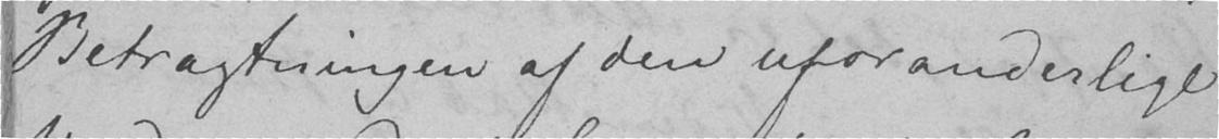Betragtningen af den uforanderlige
